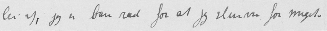lei af, jeg er bare red for at jeg slurver for meget.
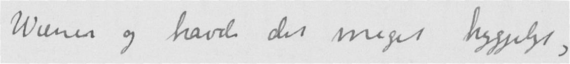Wiener og havde det meget hyggeligt,
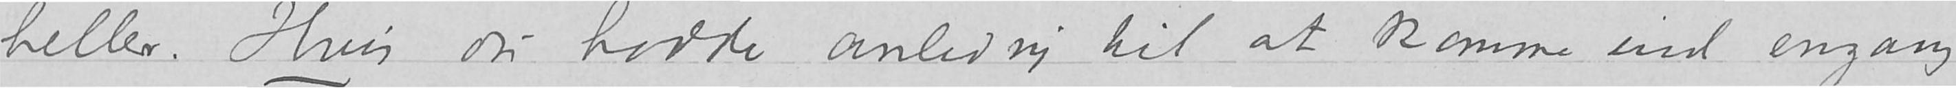heller.Hvis du hadde anledning til at komme ind engang
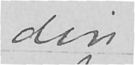din
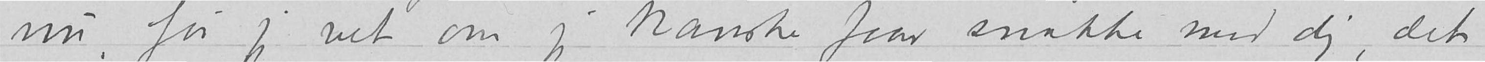nu, før jeg vet om jeg kanske faar snakke med dig, det
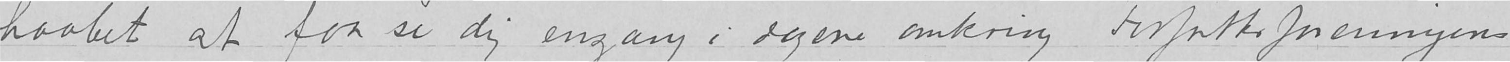haabet at faa se dig engang i dagene omkring Forfatterforeningens
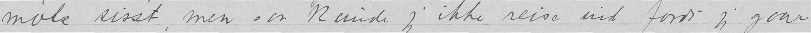møte sisst, men saa kunde jeg ikke reise ind fordi jeg gaar
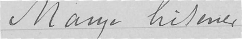Mange hilsener
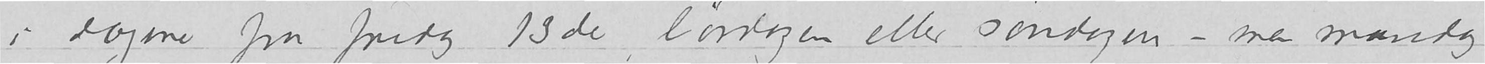i dagene fra fredag 13de, lørdagen eller søndagen - men mandag
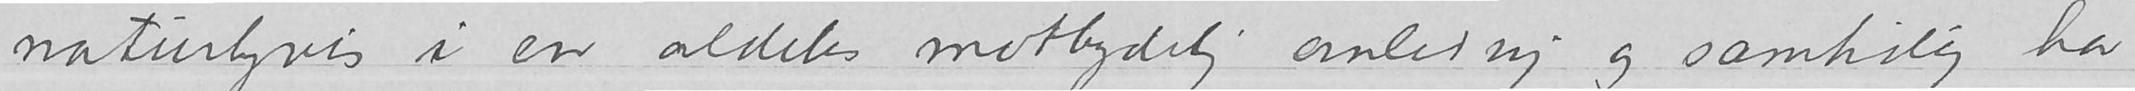naturligvis i en aldeles motbydelig anledning og samtidig har
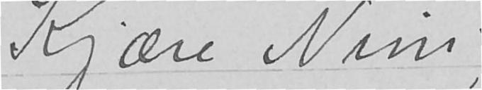Kjære Nini
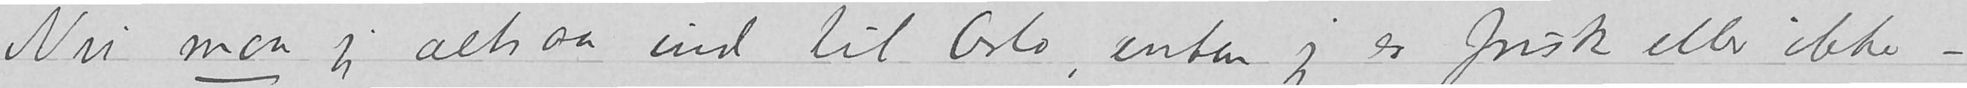Nu maa jeg altsaa ind til Oslo, enten jeg er frisk eller ikke -
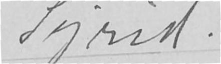Sigrid
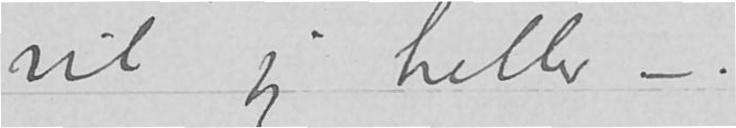vil jeg heller -.
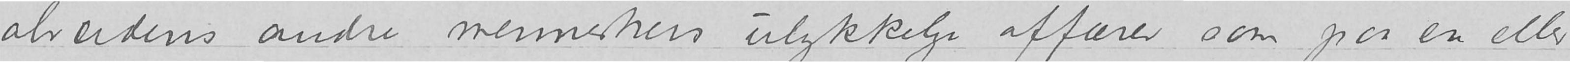og alverdens andre menneskers ulykkelige affærer som paa en eller
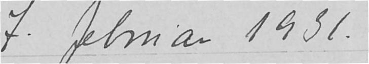7. februar 1931
